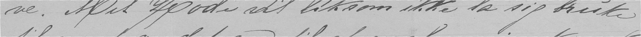ve. Mit Hode vil liksom ikke la sig bruke
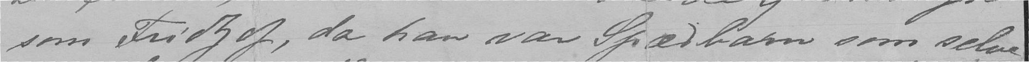som Fridtjof, da han var Spædbarn som selve-
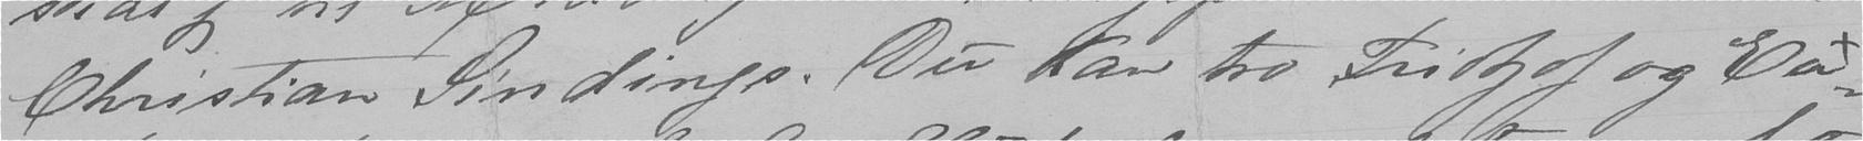Christian Sindings. Du kan tro Fridtjof og Eu-
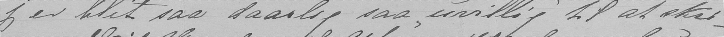jeg er blit saa daarlig saa "uvillig" til at skri-
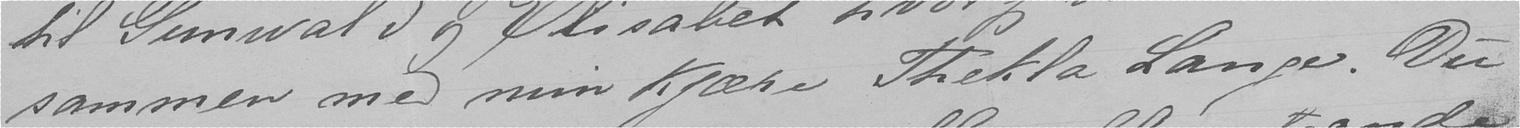sammen med min kjære Thekla Lange. Du
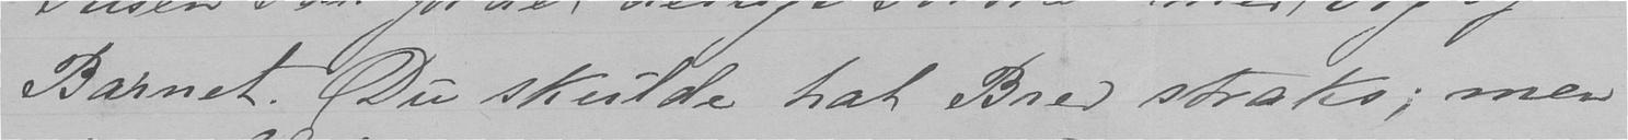Barnet. Du skulde hat Brev straks; men
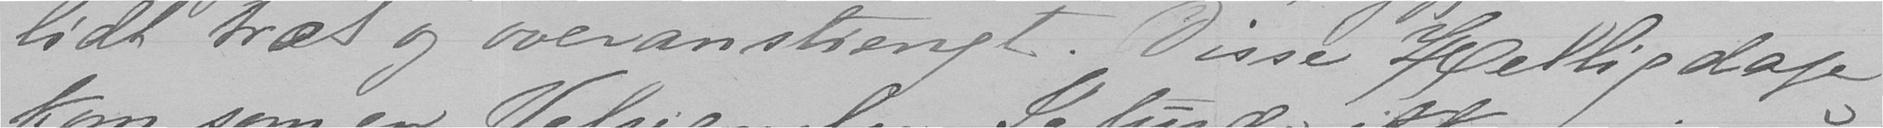lidt træt og overanstrengt. Disse Helligdage
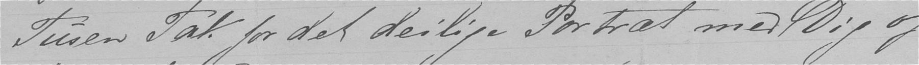Tusen Tak for det deilige Portræt med Dig og
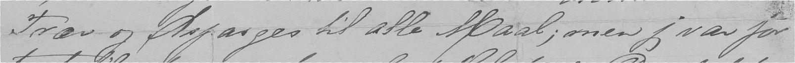Trær og Asparges til alle Maal; men jeg var for
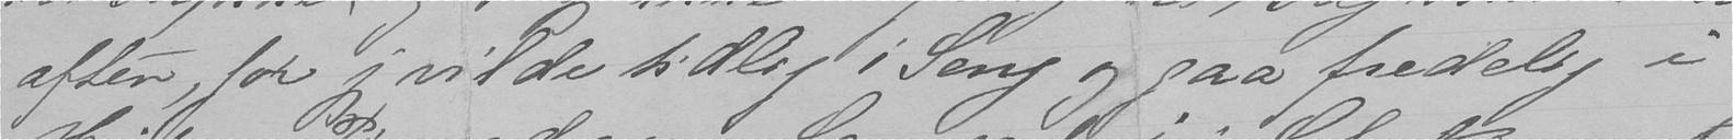aften, for jeg vilde tidlig i Seng og gaa fredelig i
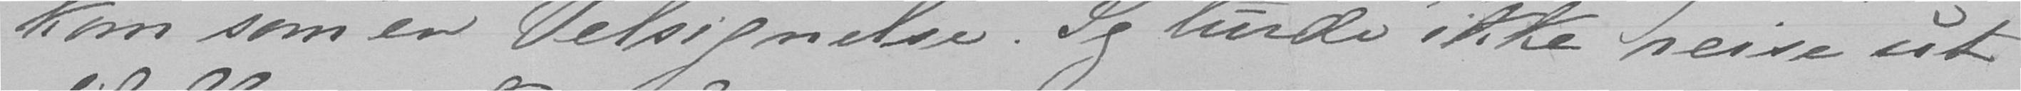kom som en Velsignelse. Jeg burde ikke reise ut
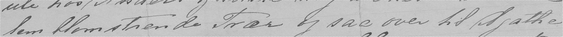lem blomstrende Trær og saa over til Agathe
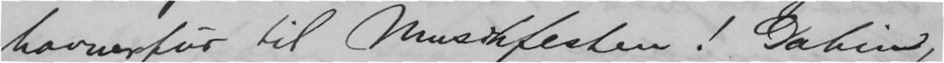havnertur til Musikfesten! Dahin,
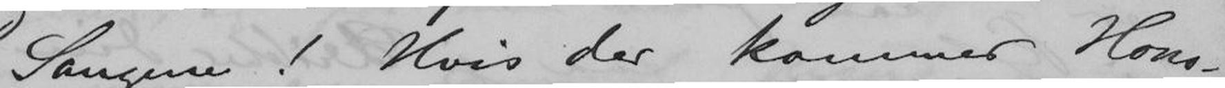Sangene! Hvis der kommer Hono-
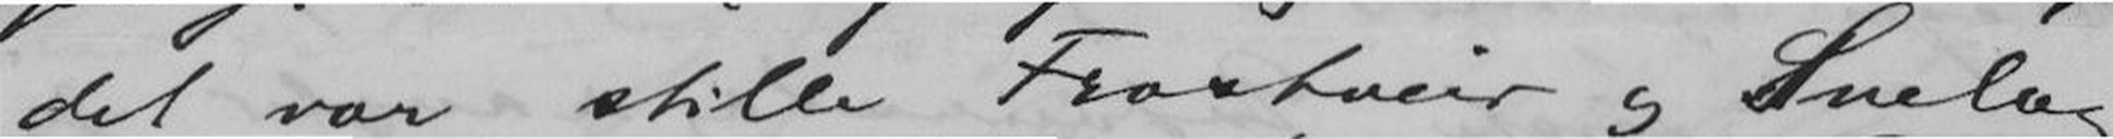det var stille Frostveir og Snelag
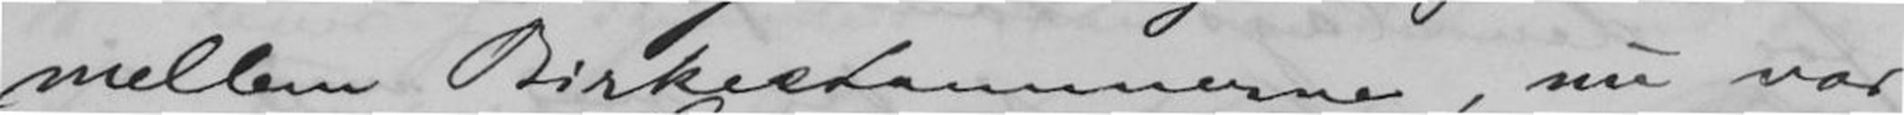mellem Birkestammerne, nu var
